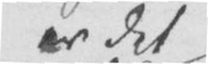er det
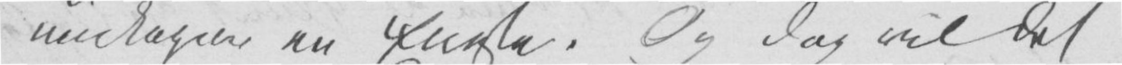undtagen en Eneste. Og dog vil det
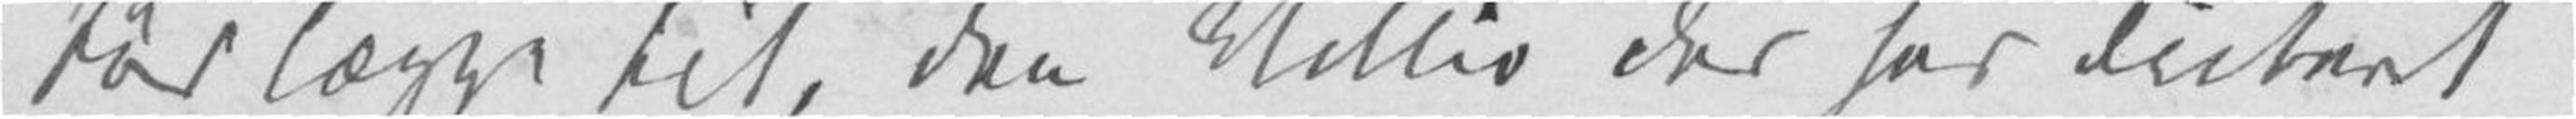tør lægge til, den Villie der har dicteret
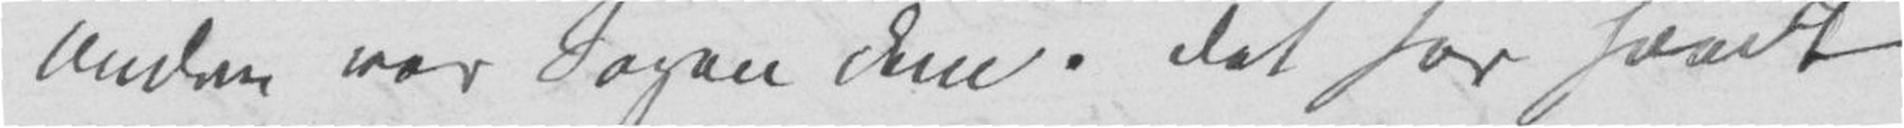anden var Sagen dem. Det har hændt
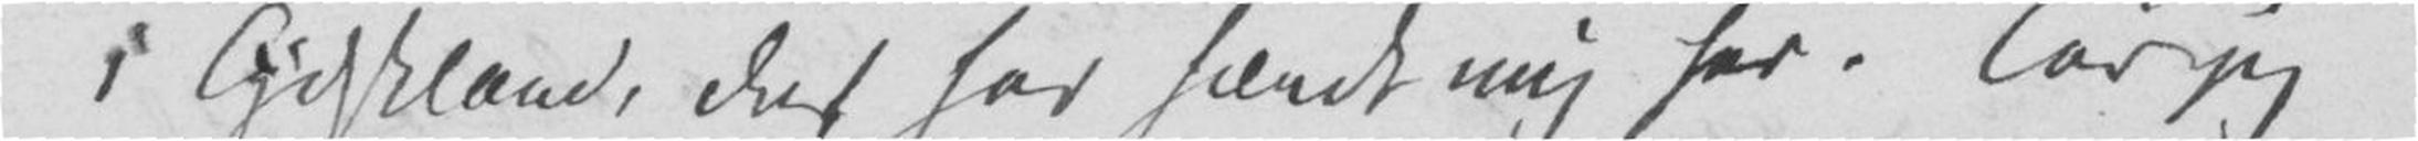i Tydskland, det har hændt mig her. Tør jeg
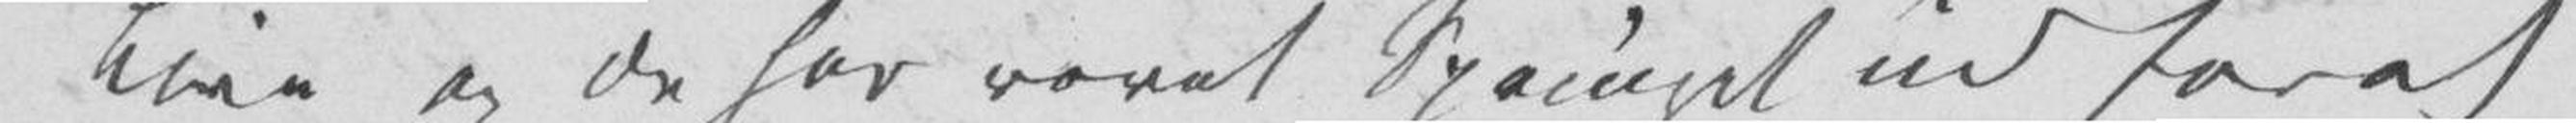Live og de har vovet Spranget ud for at
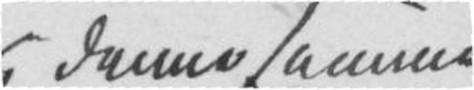i denne samme
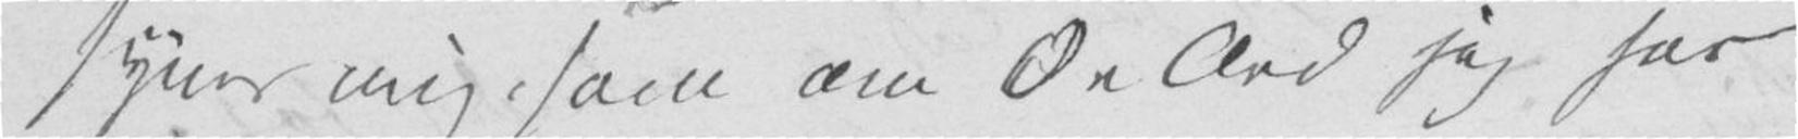synes mig, som om O<gap /> Ord jeg har
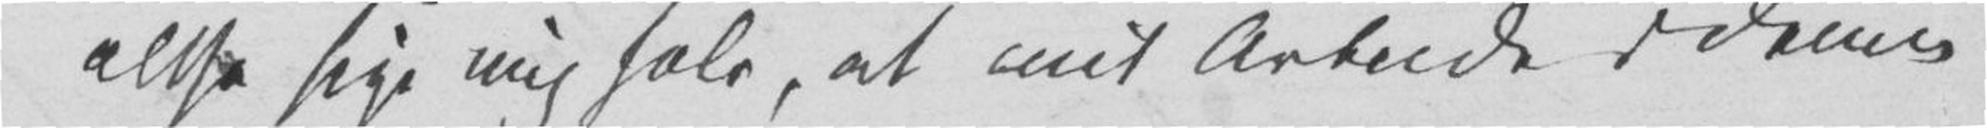altså sige mig selv, at mit Arbeide i denne
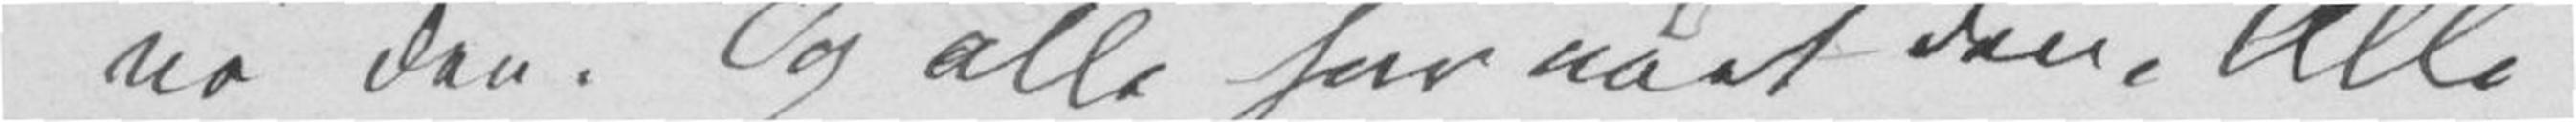nå den. Og alle har nået den. Alle
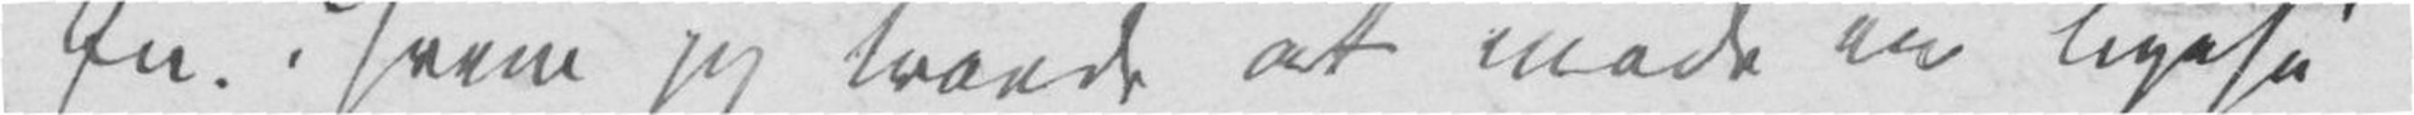En, hvem jeg troede at møde en ligeså
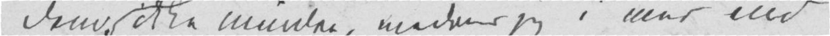dem, ikke mindre, medens jeg i mer end
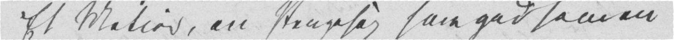Et Metier, en Pengesag saa god som en
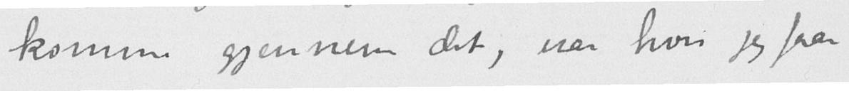komme gjennem det, især hvis jeg faar
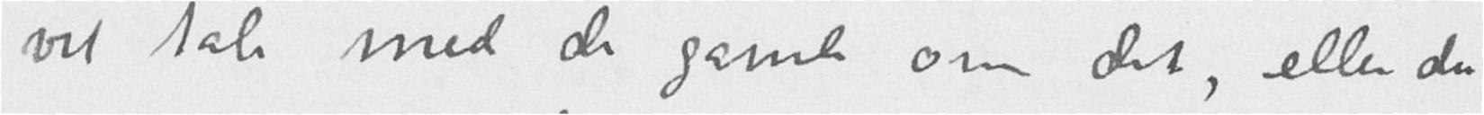vil tale med de gamle om det, eller du
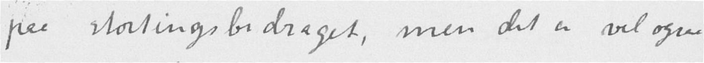paa stortingsbidraget, men det er vel ogsaa
